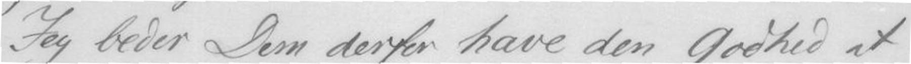Jeg beder Dem derfor have den godhed at
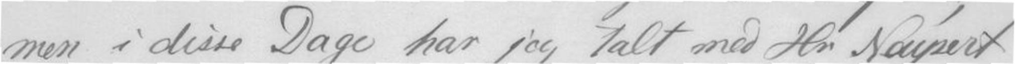men i disse Dage har jeg talt med Hr. Neupert
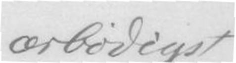ærbødigst
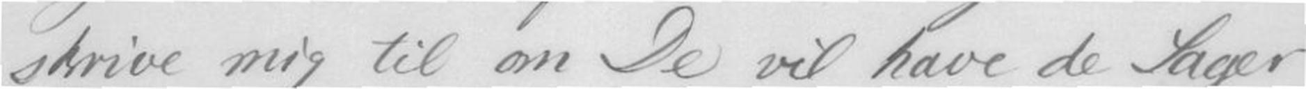skrive mig til om De vil have de Sager
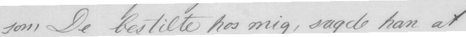som De bestilte hos mig, sagde ham at
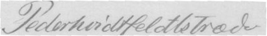Pederhvidtfeldtstræde
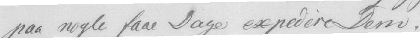paa nogle faae Dager expedere Dem.
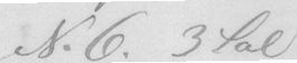N.6. 3 Sal
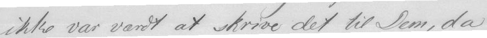ikke var værdt at skrive det til Dem, da
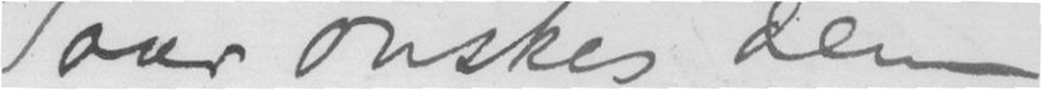Tour ønskes dem
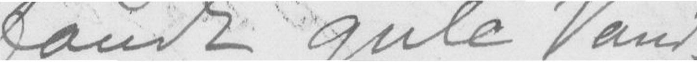fandt gule Van-
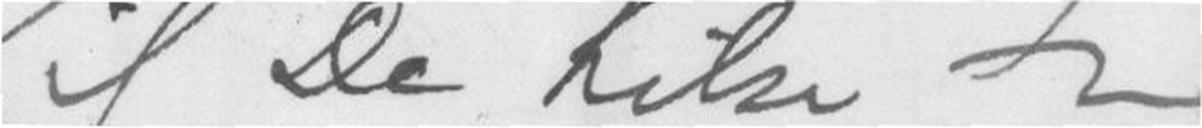Vil De hilse Fru
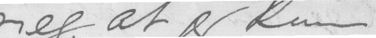Grieg, at jeg kun
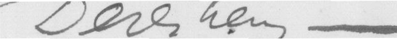Deres hengivne
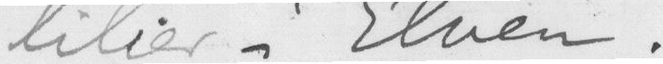lilier i Elven.
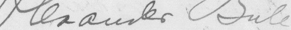Alexander Bull
